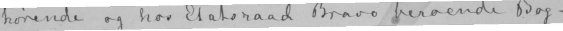hørende og hos Etatsraad Bravo beroende Bog-
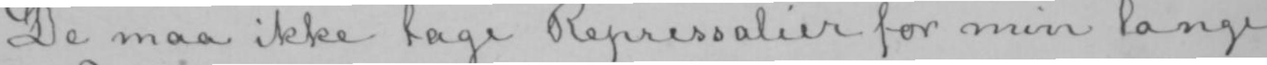De maa ikke tage Repressalier for min lange
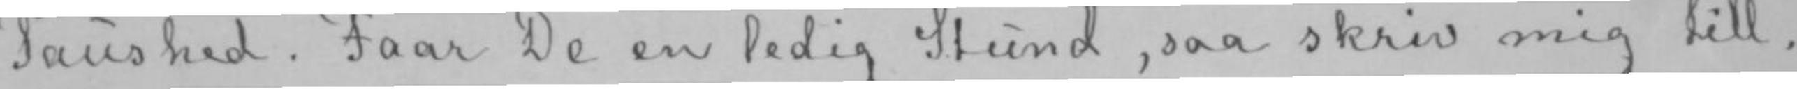Taushed. Faar De en ledig Stund, saa skriv mig till.
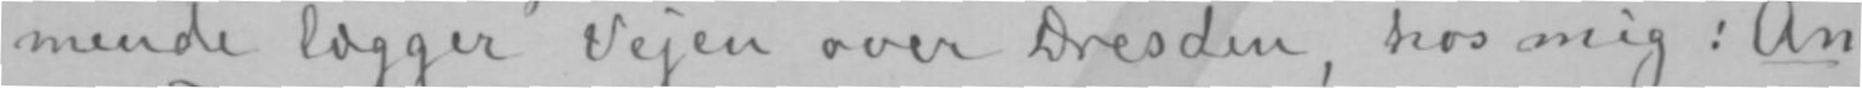mende lægger Vejen over Dresden, hos mig: An
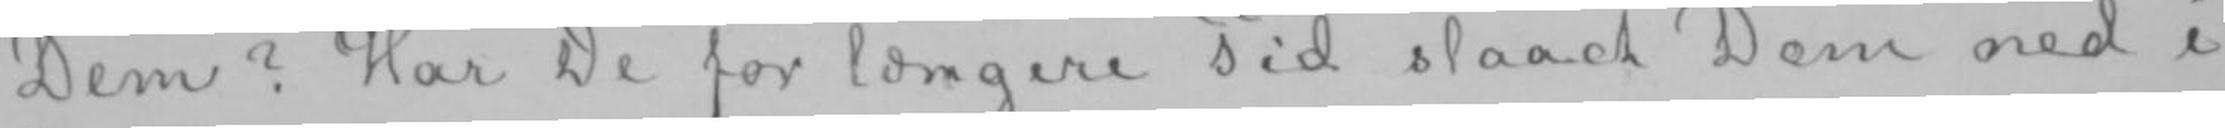Dem? Har De for længere Tid slaaet Dem ned i
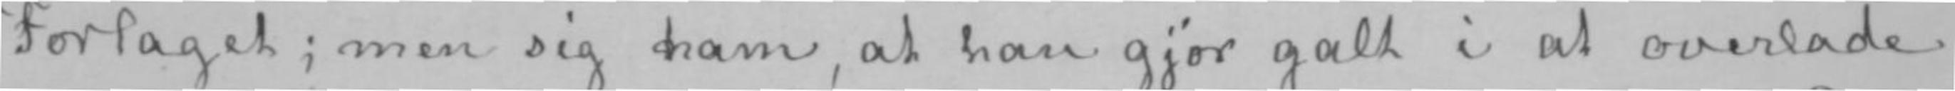Forlaget; men sig ham, at han gjør galt i at overlade
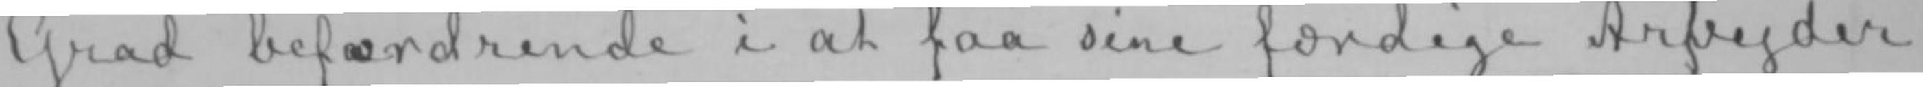Grad befordrende i at faa sine færdige Arbejder
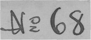No 68
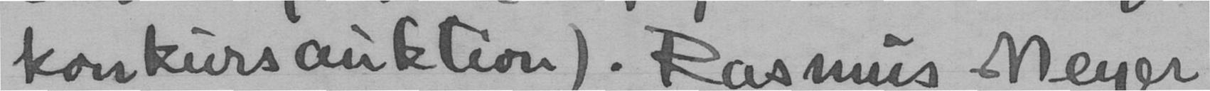konkurs auktion). Rasmus Meyer
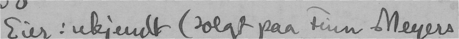Eier: ukjendt (solgt paa Finn Meyers
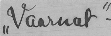"Vaarnat"
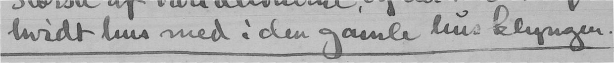hvidt hus med i den gamle hus klyngen.
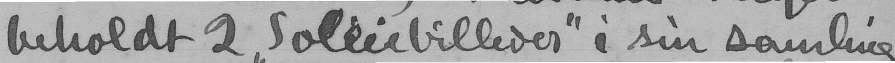beholdt 2 "Soleiebillider" i sin samling
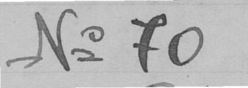No 70
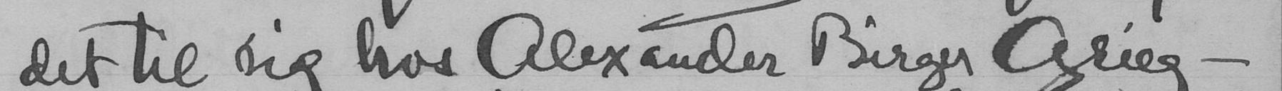det til sig hos Alexander Birger Grieg -
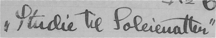"Studie til Soleienatten"
